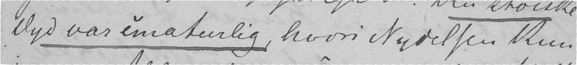Dyd var unaturlig, hvori Nydelsen kun
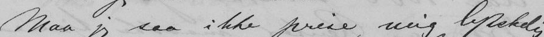Maa jeg saa ikke prise mig lykkelig
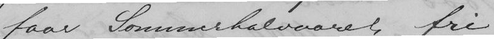faar Sommerhalvaaret fri
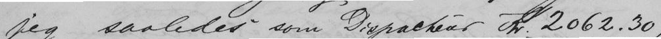jeg saaledes som Dispacheur Kr. 2062.30,
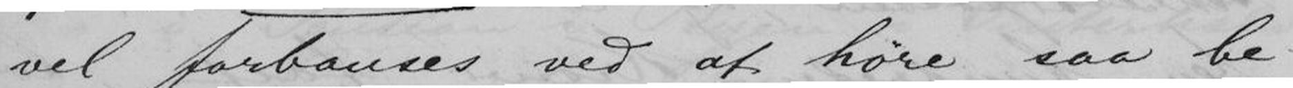vel forbauses ved at høre saa be-
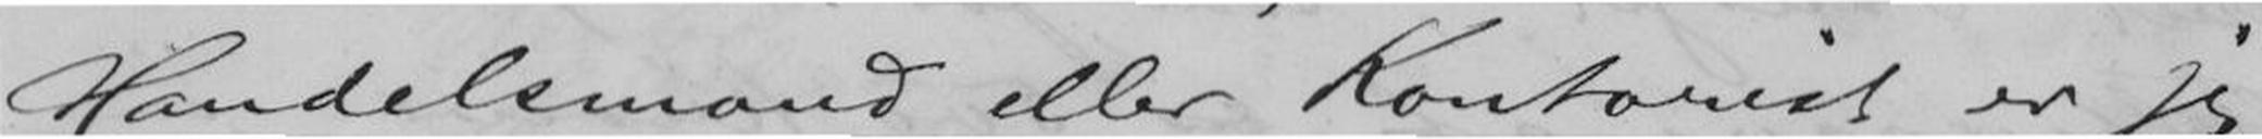Handelsmand eller Kontorist er jeg
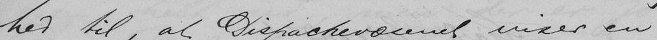hed til, at Dispachevæsenet viser en
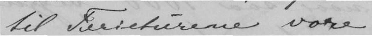til Ferieturene vore,
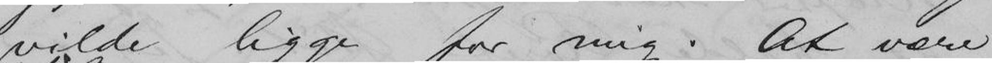vilde ligge for mig. At være
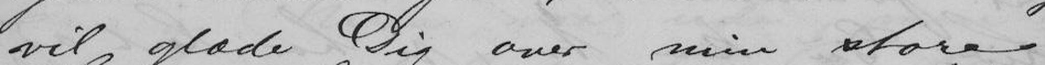vil glæde Dig over min store
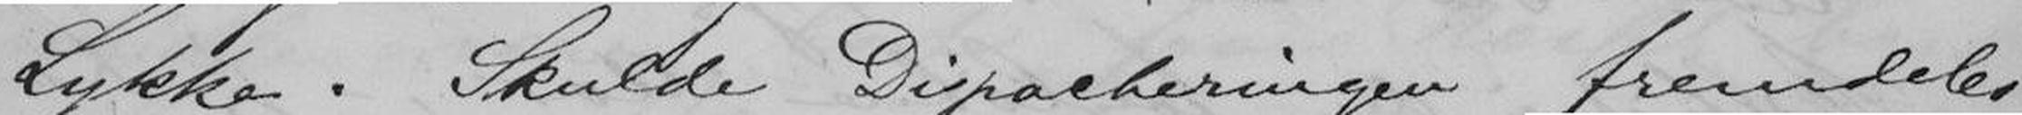Lykke. Skulde Dispacheringen fremdeles
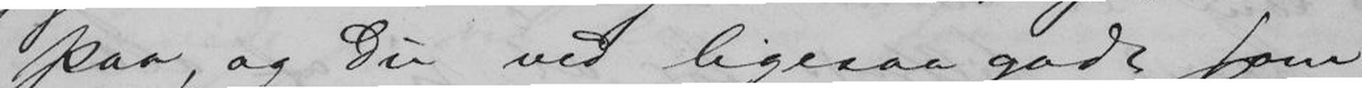paa, og Du ved ligesaa godt som
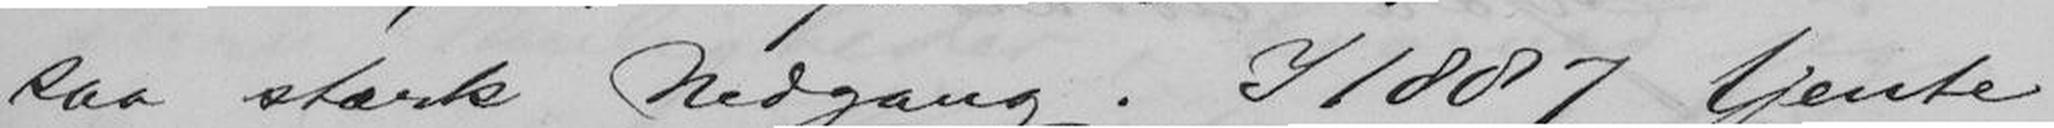saa stærk Nedgang. I 1887 tjente
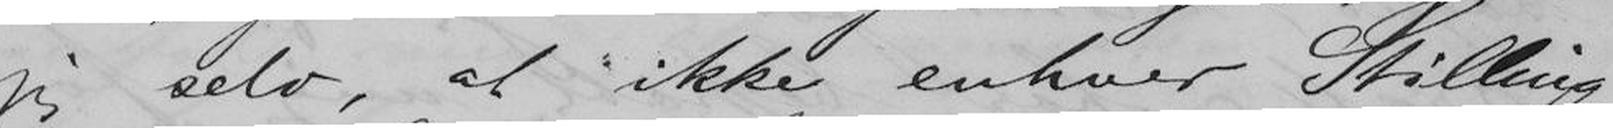jeg selv, at ikke enhver Stilling
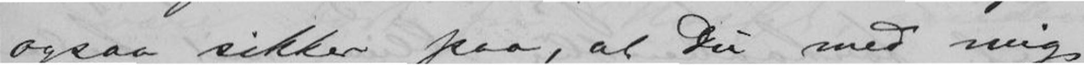ogsaa sikker paa, at Du med mig
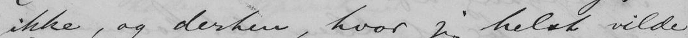ikke, og derhen, hvor jeg helst vilde
